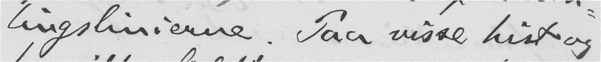lingslinierne. Paa visse hist og
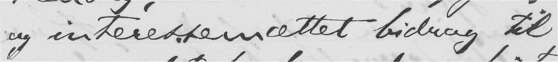og interessemættet bidrag til
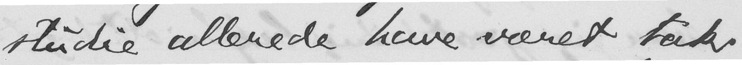studie allerede have været tak
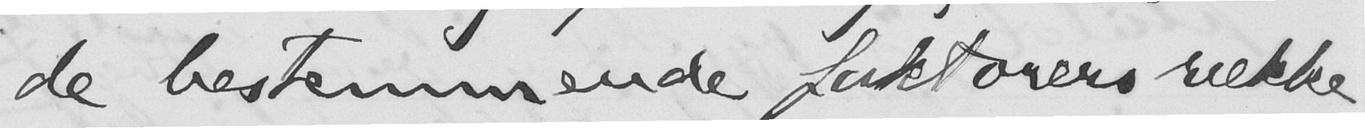de bestemmende faktorers række
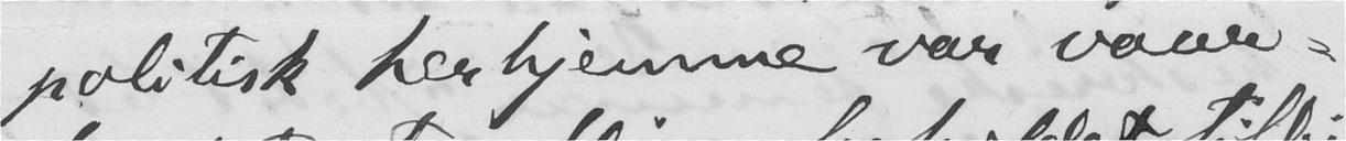politisk herhjemme var vaar-
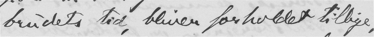brudets tid, bliver forholdet tillige,
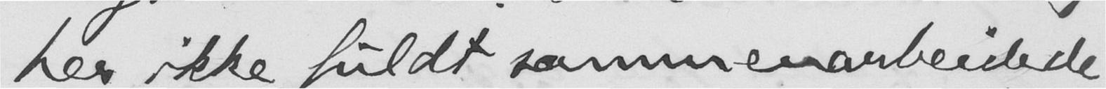her ikke fuldt sammenarbeidede
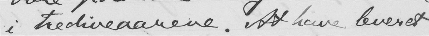i trediveaarene. At have leveret
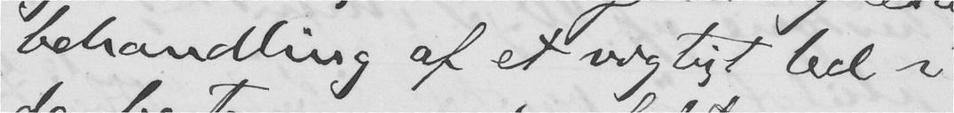behandling af et vigtigt led i
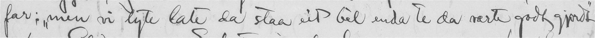far: "men vi lyte late da staa eit bil enda te da varte godt gjordt"
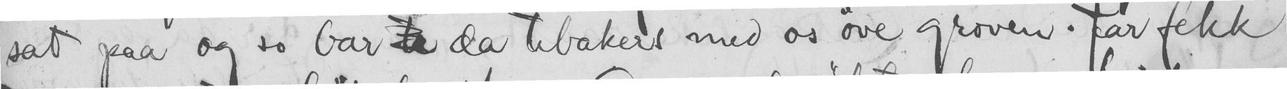sat paa og so bar  da tebakers med os øve groven. Far fekk
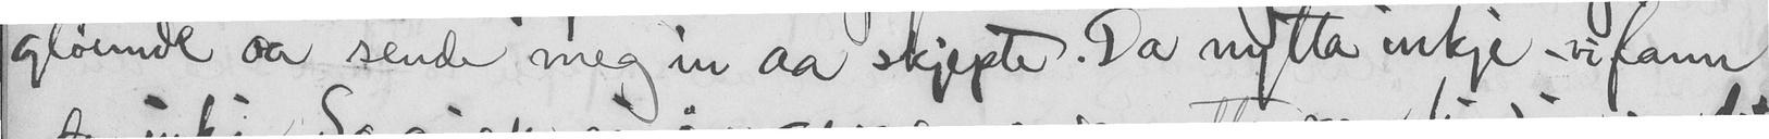gløimde aa sende meg in aa skjepte. Da nytta inkje, vi fann
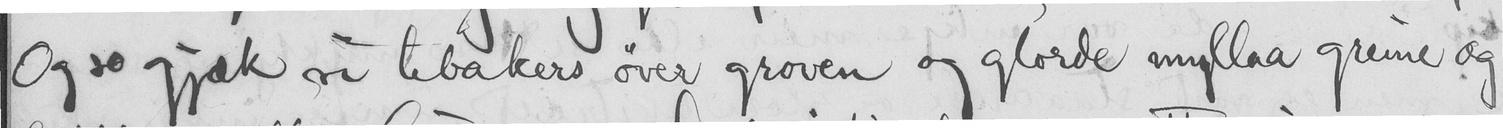Og so gjæk vi tebakers øver groven og glorde myllaa greine og
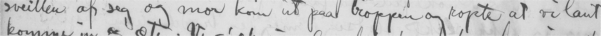sveitten af seg og mor kom ut paa troppen og ropte at vi laut
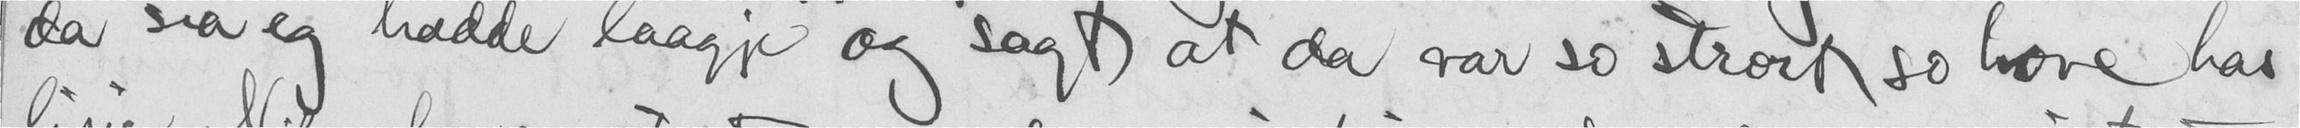da sia og hadde laagje og sagt at da var so stort so have har
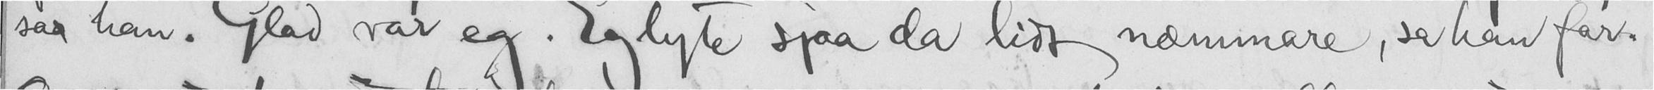sa han. Glad var eg. Eg lyte sjaa da lidt næmmare, sa han far.
